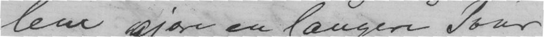lem gjøre en længere Tour
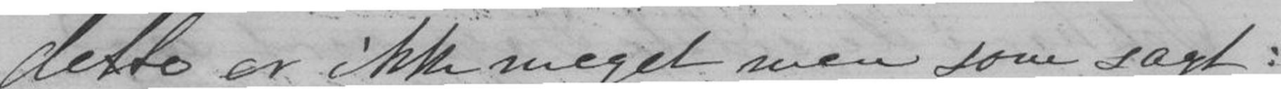dette er ikke meget, men som sagt:
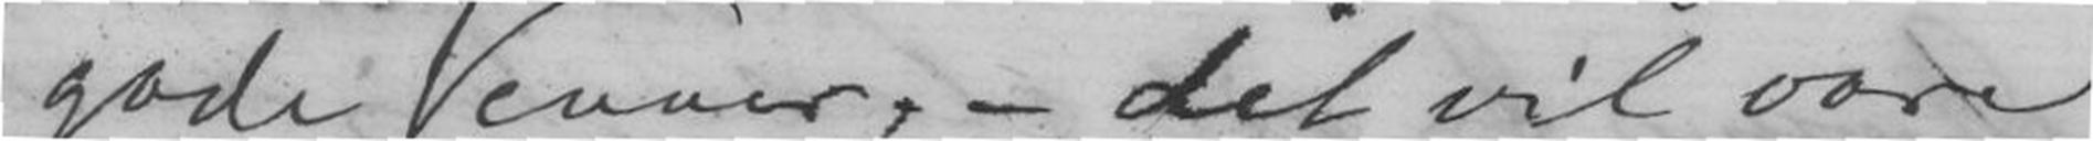gode Venner, - det vil vore
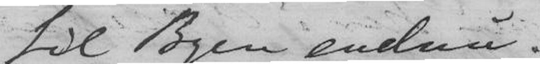til Byen endnu.
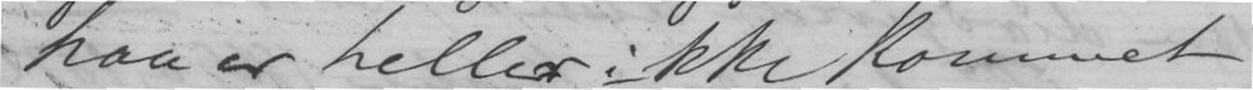han er heller ikke kommet
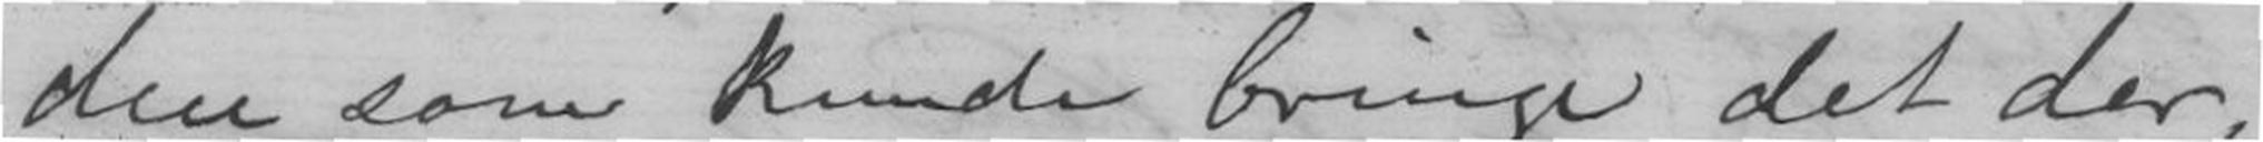den som kunde bringe det der,
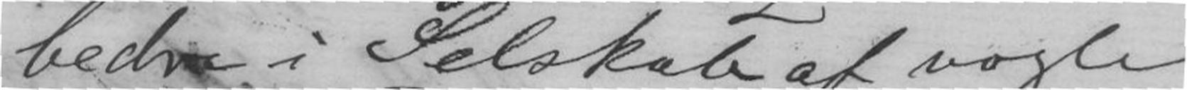bedre i Selskab af nogle
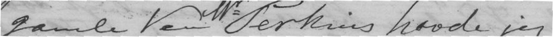gamle Ven Mr Perkins havde jeg
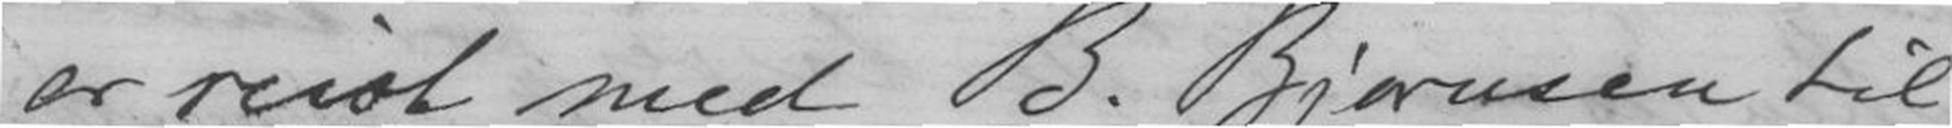er reist med B. Bjørnsen til
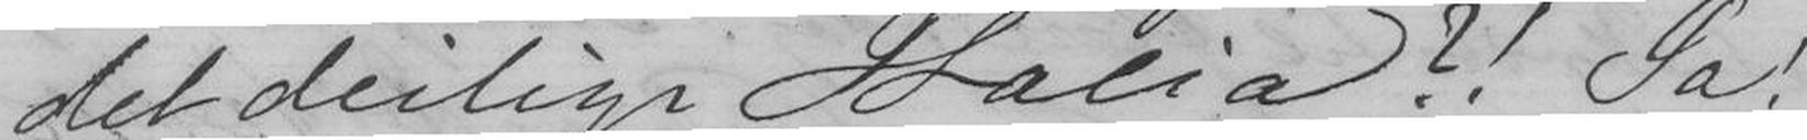det deilige Italia?! Ja!
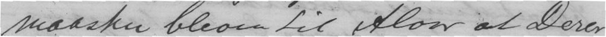maaske bleven til Alvor at Derer
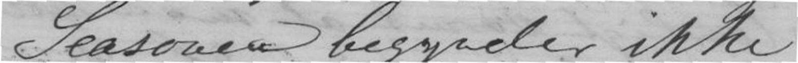Seasonen begynder ikke
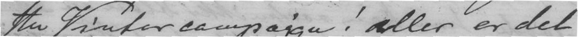thi Vinter campajgu! eller er det
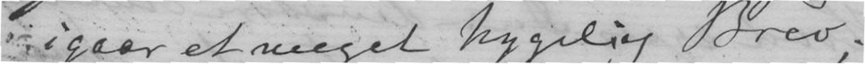igaar et meget hygelig Brev;
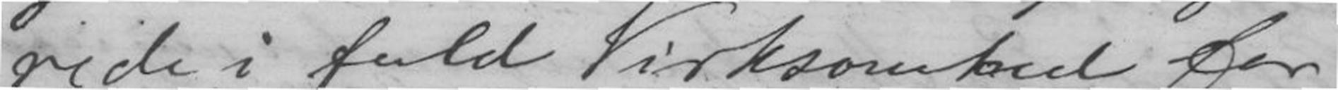rede i fuld Virksomhed for
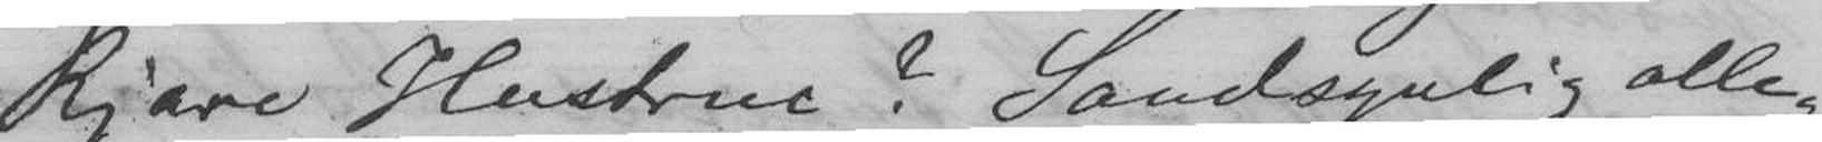kjære Hustru? Sandsynlig alle.
